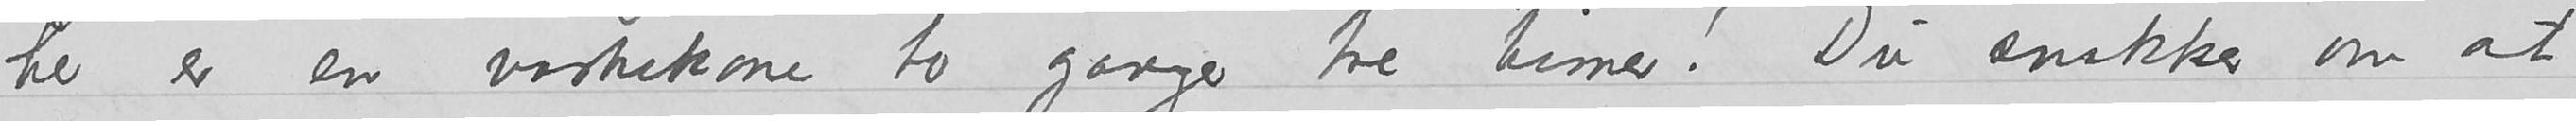her er en vaskekone to ganger tre timer! Du snakker om at
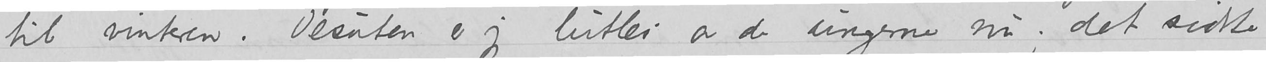til vinteren. Desuten er jeg lutlei av de ungerne nu; det sidste
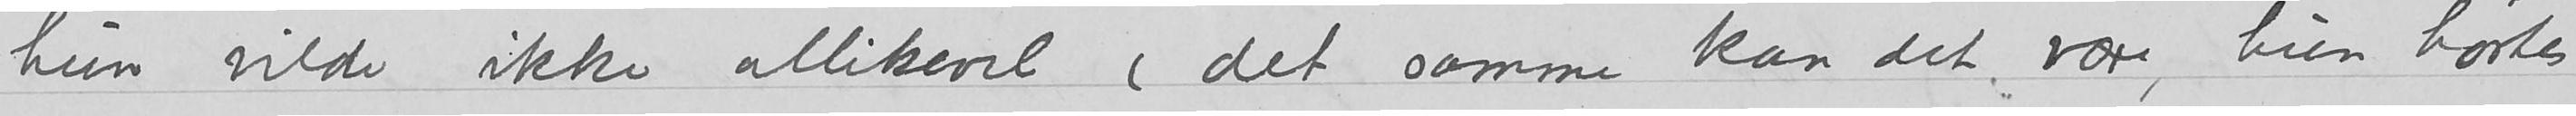hun vilde ikke allikevel (det samme kan det være, hun hørtes
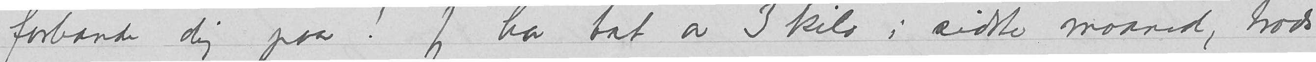forbande dig paa! Jeg har tat av 3 kilo i sidste maaned, trods
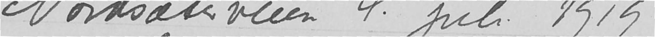Nordsæterveien  4. juli 1919
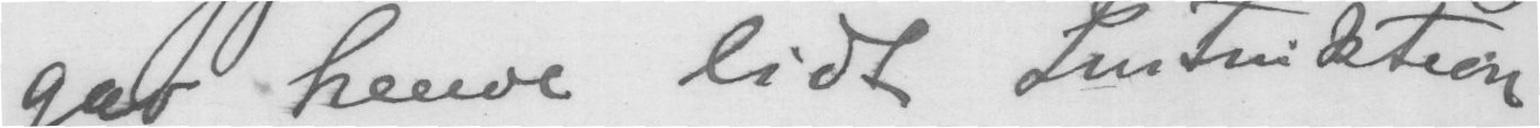gav hende lidt Instruktion
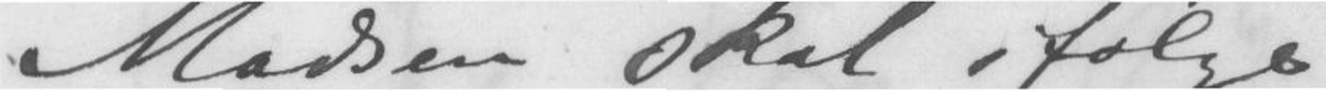Madsen skal ifølge
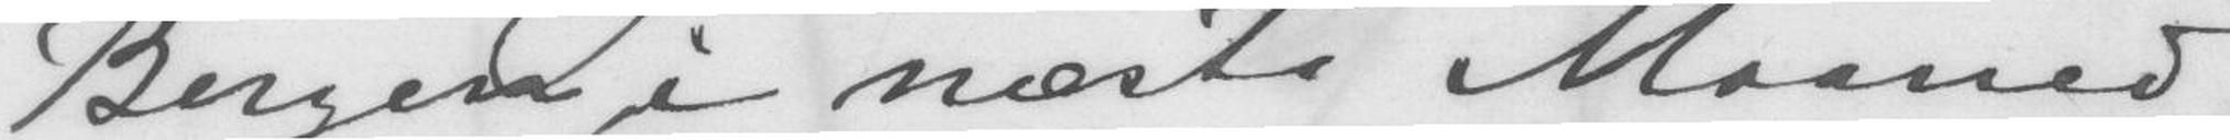Bergen i næste Maaned
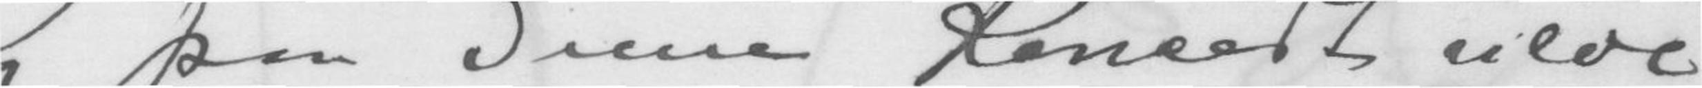og paa denne koncert vilde
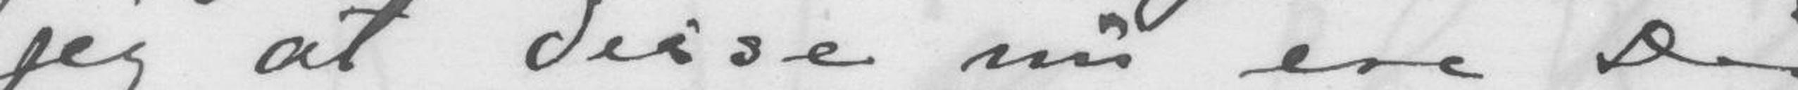jeg at disse nu ere Dig
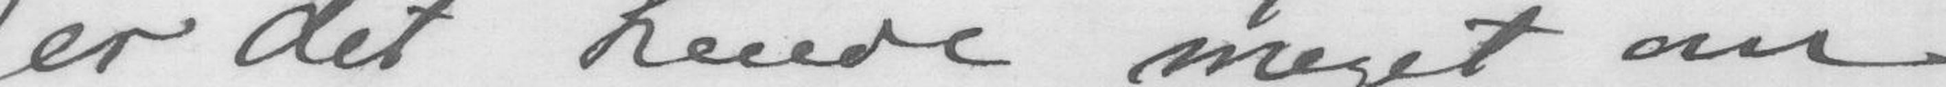er det hende meget om
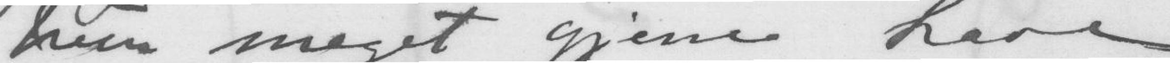hun meget gjerne have
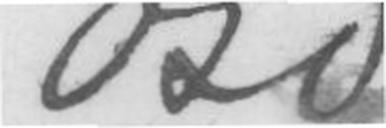osv
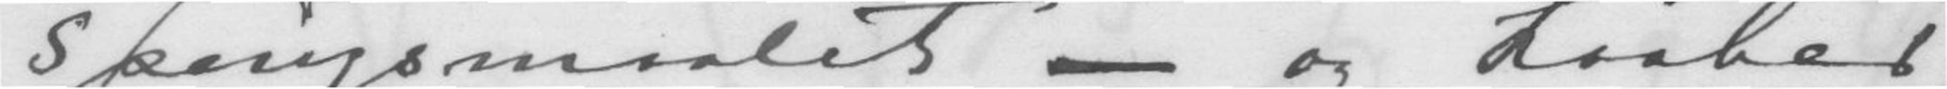spørgsmaalet - og haaber
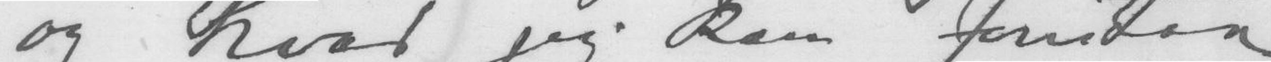og hvad jeg kan forstaa
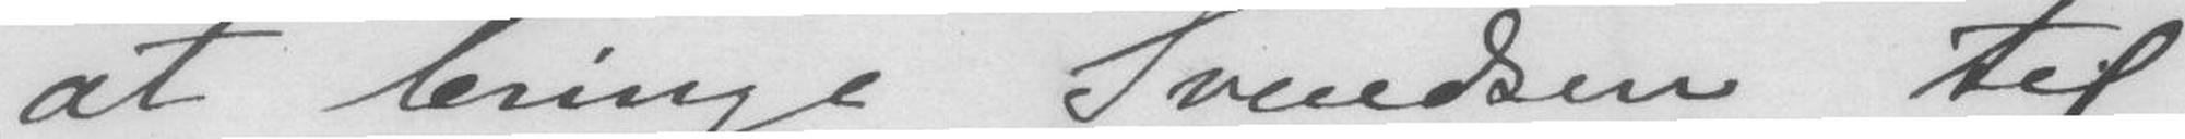at bringe Svendsen til
